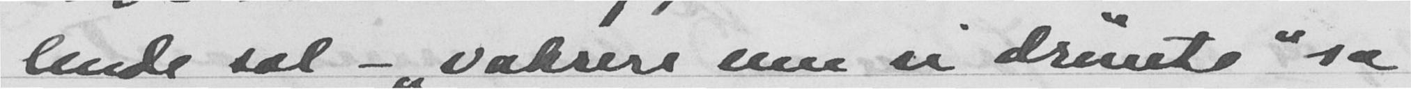lende sol - "vakrere enn vi drømte" sa
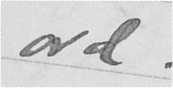ord.
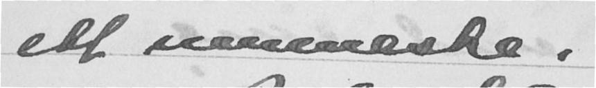ett menneske.
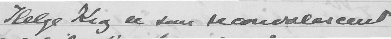Helge Krog er som teconvalescend
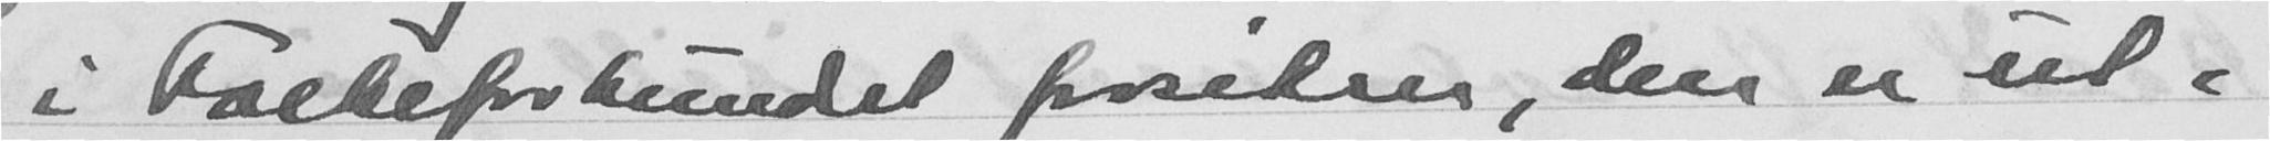i Folkeforbundet forsiden, den er ut-
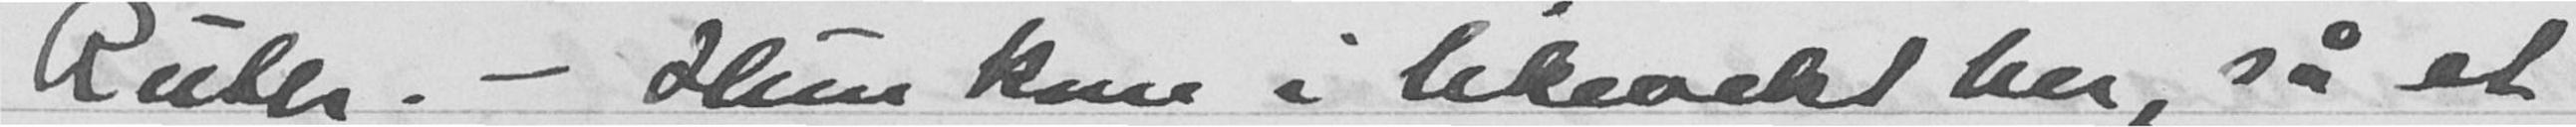Ruth. - Hun kom i likevekt her, så et
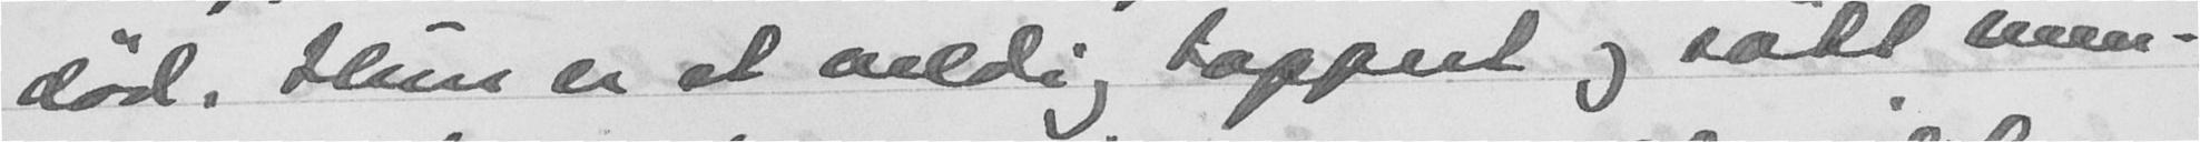død. Hun er et veldig tappert og søtt men-
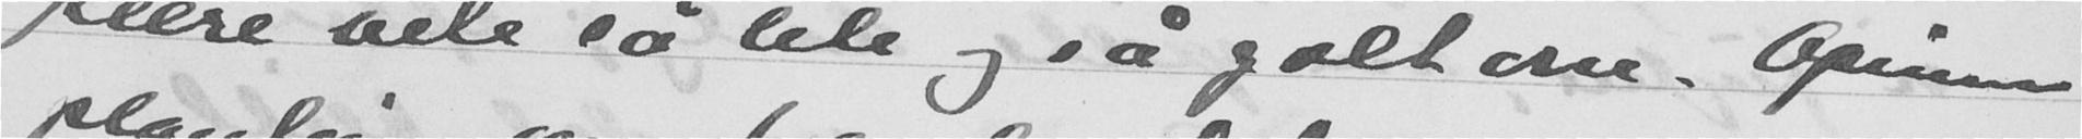kcere vete så lite og så galt om. Opium
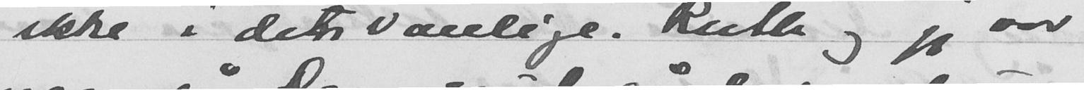ikke i det vanlige. Ruth og jeg var
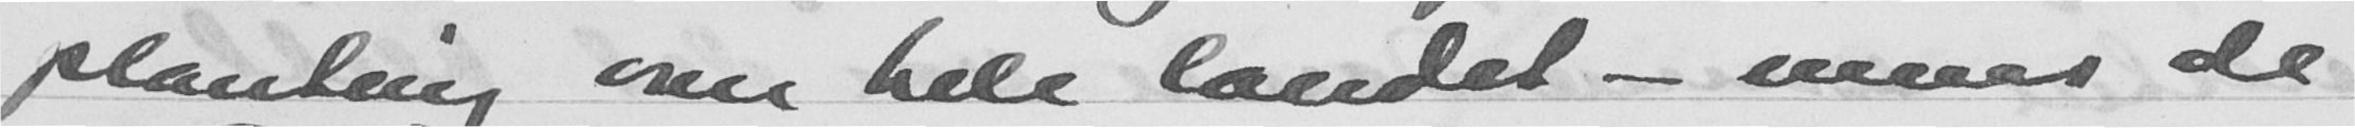planting over hele landet - mens de
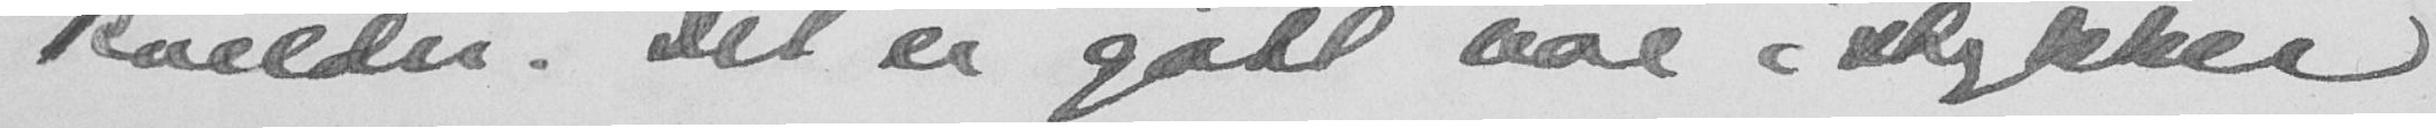kvelder. Det er gått noe istykker
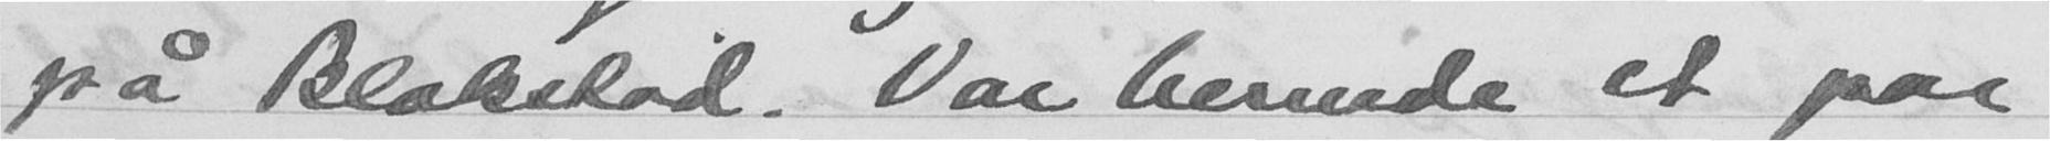på Blakstad. Var herude et par
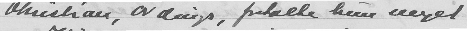Christian, Ordings, fortalte hun meget
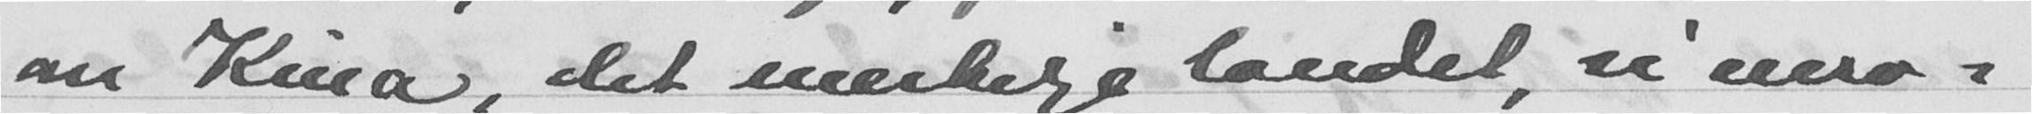om Kina, det merkelige landet, vi mro-
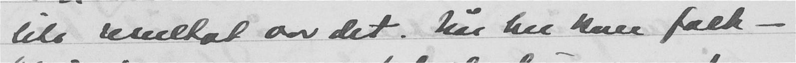lite resultat var det. Når her kom folk -
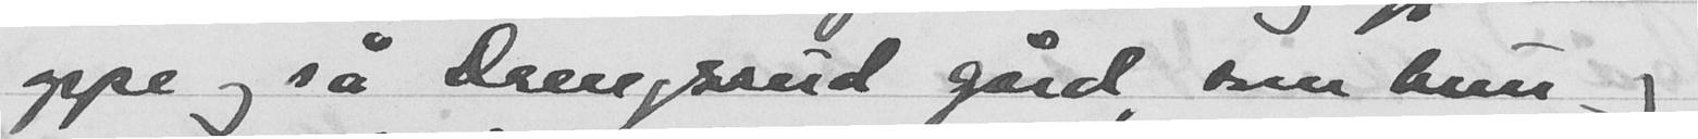oppe og sa Drengsrud gård, som hun og
